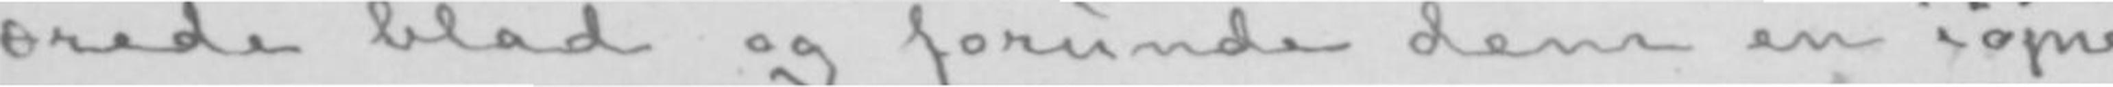ærede blad og forunde dem en iøjne-
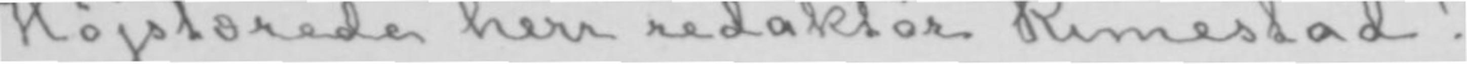Højstærede herr redaktør Rimestad!
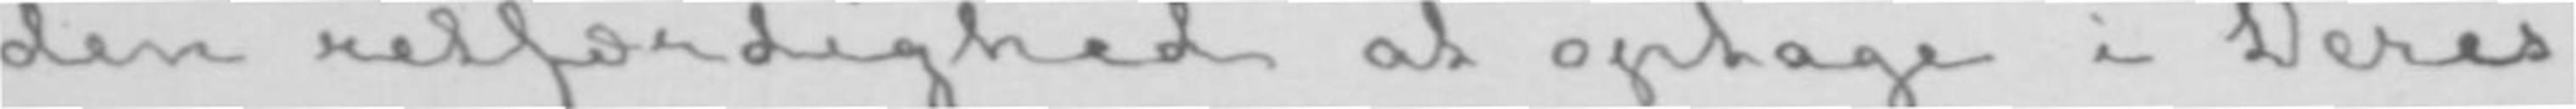den retfærdighed at optage i Deres
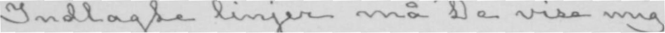Indlagte linjer må De vise mig
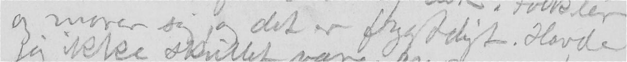og morer sig, og det er frygteligt. Havde
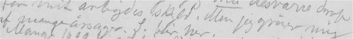for mit arbejdes skyld. Men jeg gruer mig
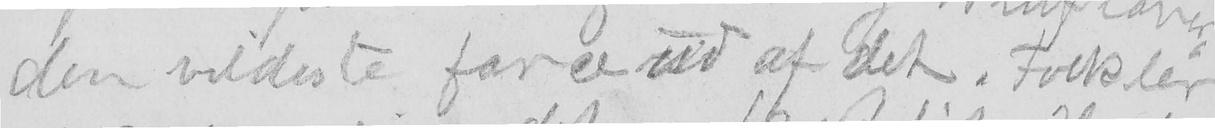den vildeste farce ud at det. Folk ler
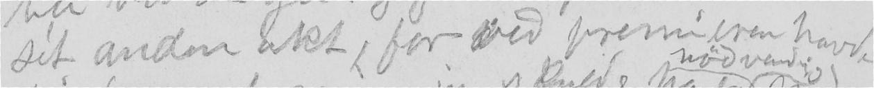sét anden akt, for ved premieren havde
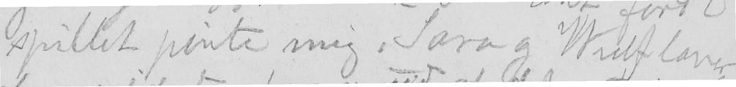spillet pinte mig. Sara og Wulf laver
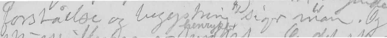forståelse og begejstring", siger man. Og
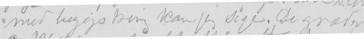med begejstring kan jeg sige. De græder
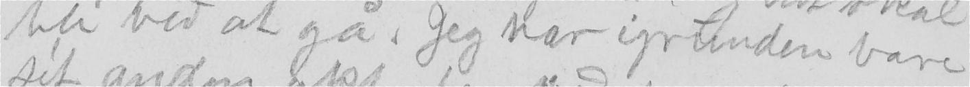bli ved at gå. Jeg har igrunden bare
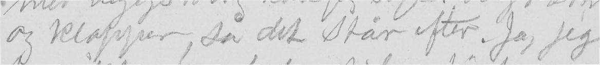og klapper så det står efter. Ja, jeg
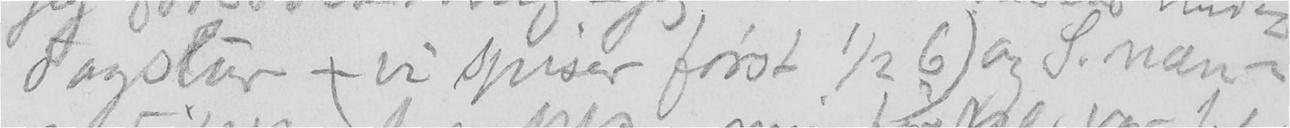dagslur - (vi spiser først 1/2 6) og S. næn-
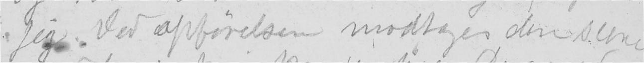jeg. Ved opførelsen modtages den scene
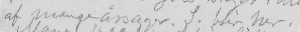af mange årsager. S. blir her.
